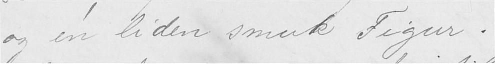og en liden smuk Figur.
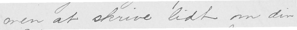men at skrive lidt om din
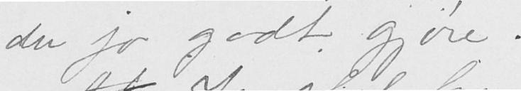du jo godt gjøre.
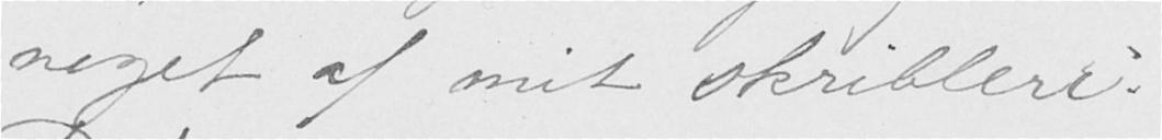noget af mit skribleri.
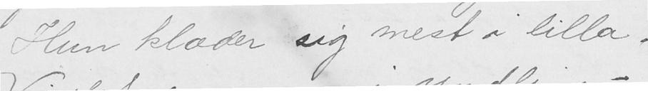Hun klæder sig mest i lilla.
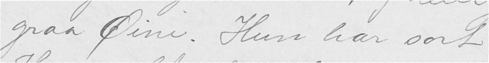graa Øine. Hun har sort
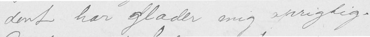dent har glæder mig oprigtig.
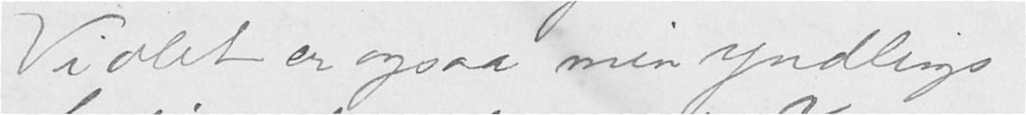Violet er ogsaa min yndlings-
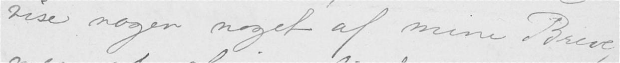vise nogen noget af mine Breve,
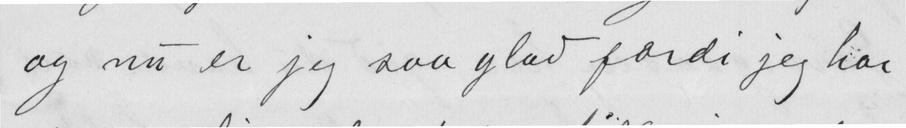og nu er jeg saa glad fordi jeg har
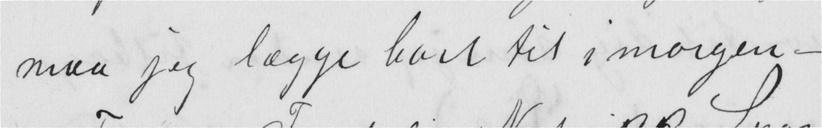maa jeg lægge bort til imorgen -
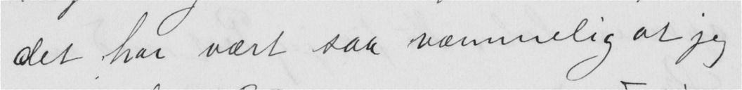det har vært saa væmmelig at jeg
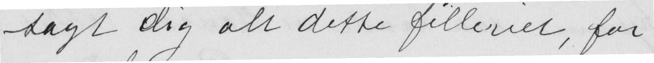sagt dig alt dette filleriet, for
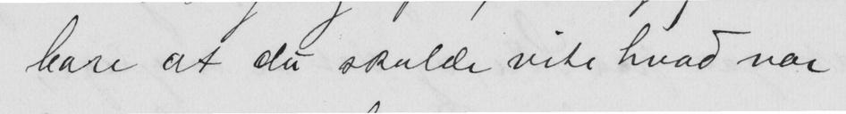bare at du skulde vite hvad var
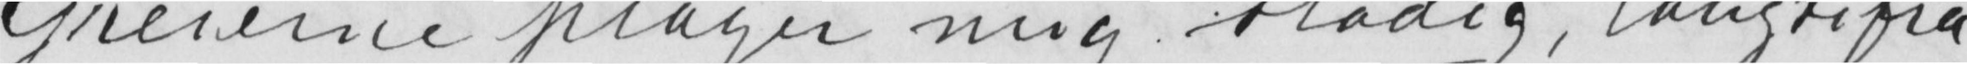Greiene plager mig stadig, langtifra
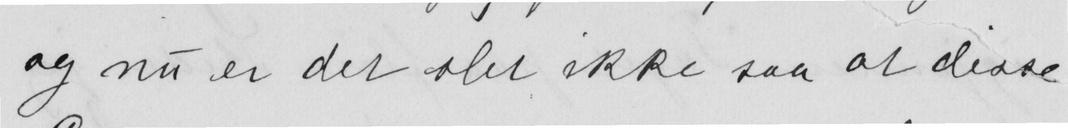og nu er det slet ikke saa at disse
